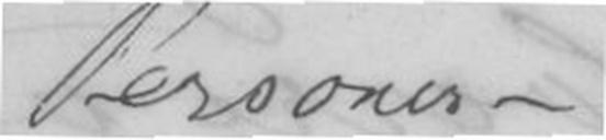Personer.
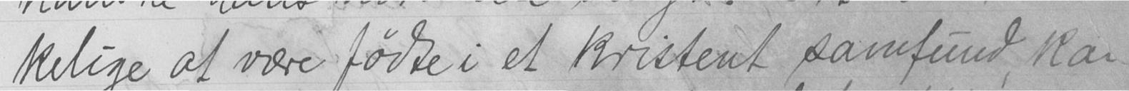kelige at være fødte i et kristent samfund, kan
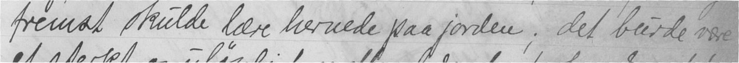fremst skulde lære hernede paa jorden; det burde være
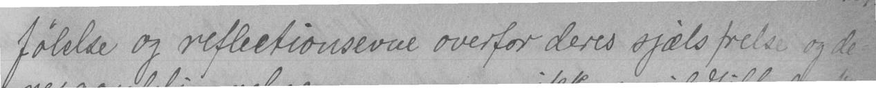følelse og reflectionsevne overfor deres sjæls frelse og de-
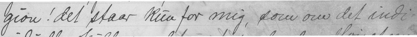gion! det staar kun for mig, som om det indi-
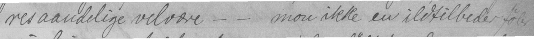res aandelige velvære - - mon ikke en ildtilbeder føler
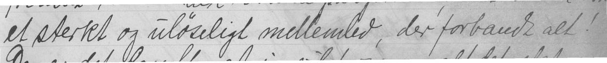et sterkt og uløseligt mellemled, der forbandt alt!
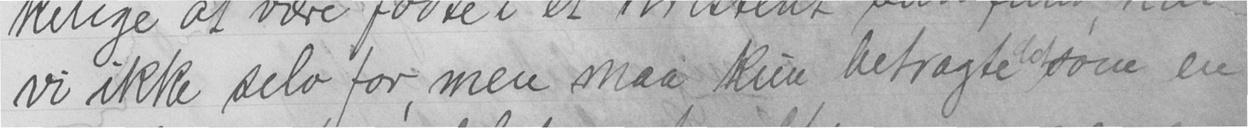vi ikke selv for, men maa kun betragte som en
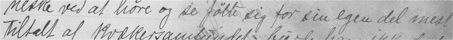neske ved at høre og se følte sig for sin egen del mest
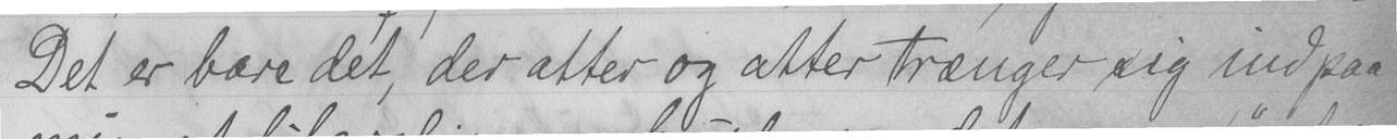Det er bare det, der atter og atter trænger sig ind paa
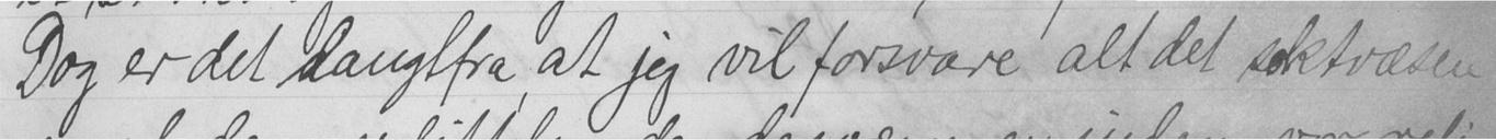Dog er det langtfra, at jeg vil forsvare alt det sektvæsen
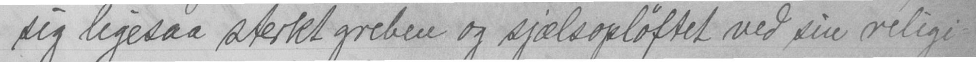sig ligesaa sterkt greben og sjælsopløftet ved sin religi-
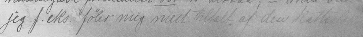jeg f. eks. føler mig mest tiltalt af den katholske
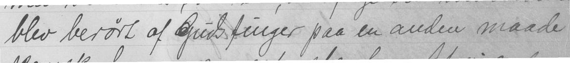blev berørt af Guds finger paa en anden maade
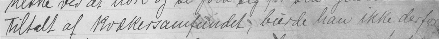tiltalt af kvækersamfundet, burde han ikke derfor
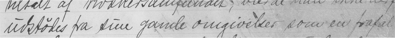udstødes fra sine gamle omgivelser som en frafal
